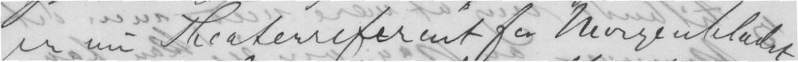er nu Theaterreferent for Morgenbladet
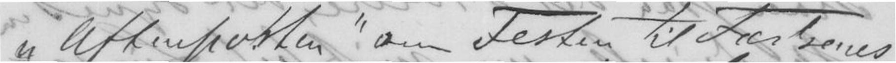"Aftenposten" om Festen til Fædrenes
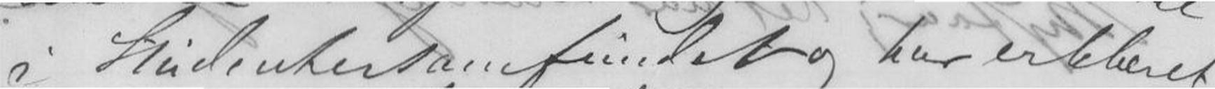i Studentersamfundet og har erklæret
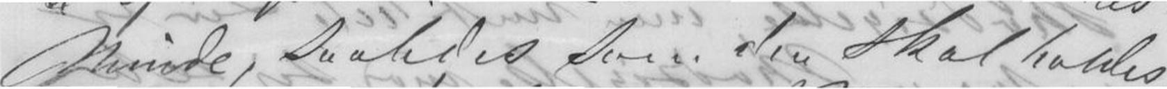Minde, saaledes som den skal holdes
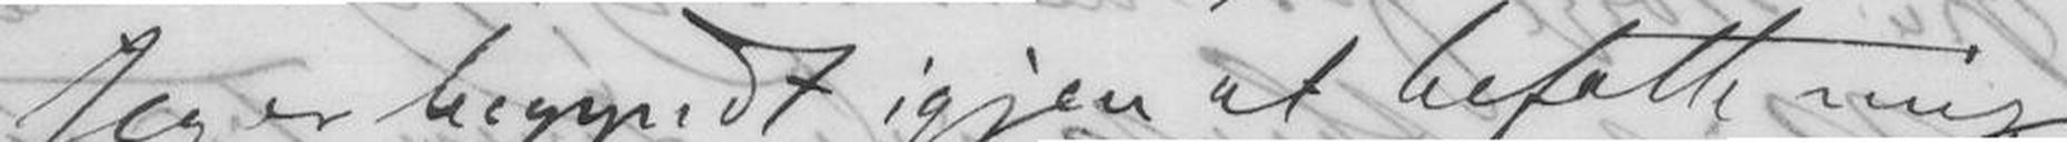Jeg er begyndt igjen at befatte mig
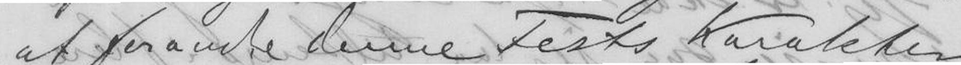at forandre denne Fests Karakter
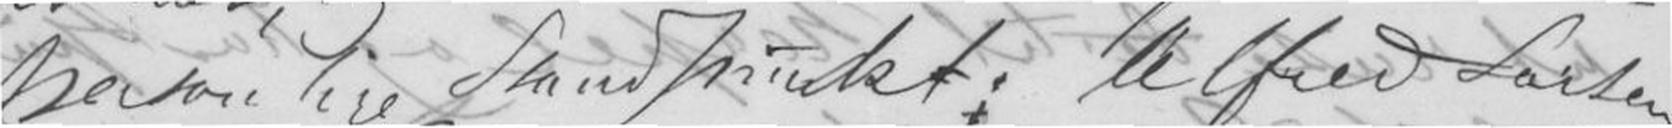personlige Standpunkt; Alfred Larsen
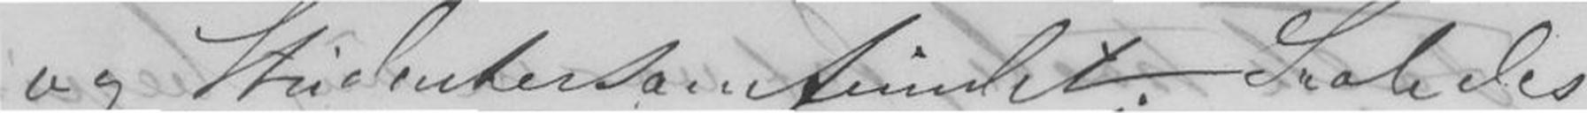og Studentersamfundet. Saaledes
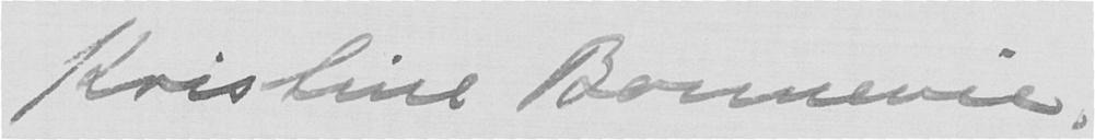Kristine Bonnevie.
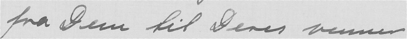fra Dem til Deres venner
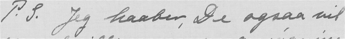P.S. Jeg haaber De ogsaa vil
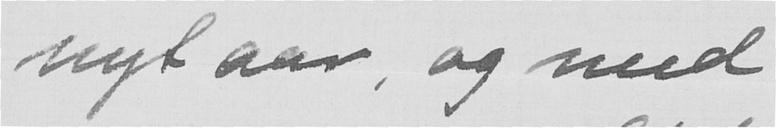nyt aar, og med
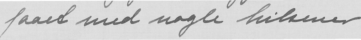faaet med nogle hilsener
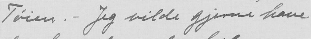Tøien.- Jeg vilde gjerne have
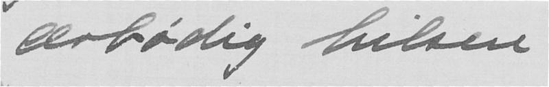ærbødig hilsen
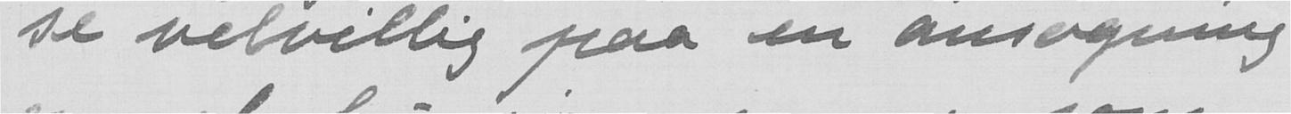se velvellig paa en ansøgning
